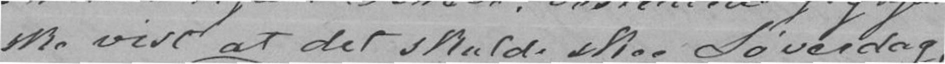ske vist at det skulde skee Løverdag,
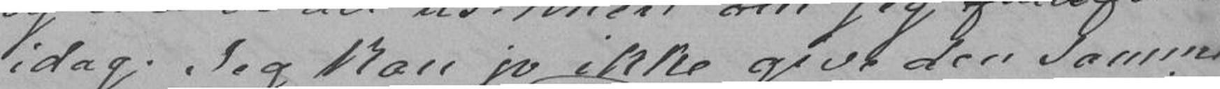idag. Jeg kan jo ikke give den samme
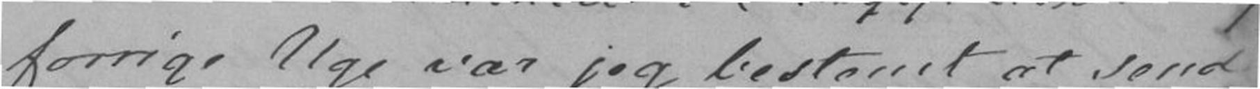forrige Uge var jeg bestemt at sende
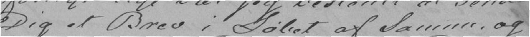Dig et Brev i Løbet af Samme, og
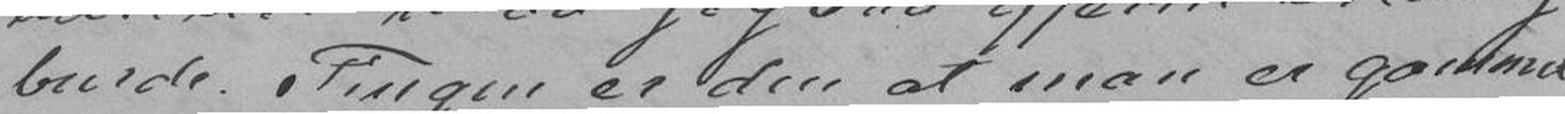burde. Tingen er den at man er gammel
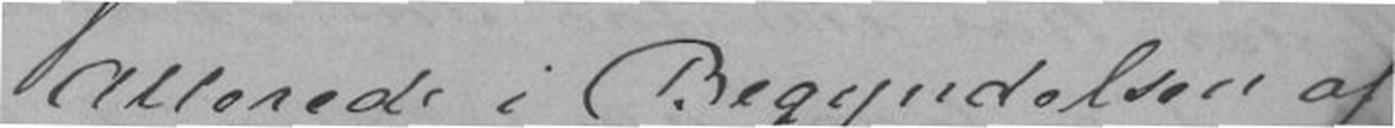Allerede i Begyndelsen af
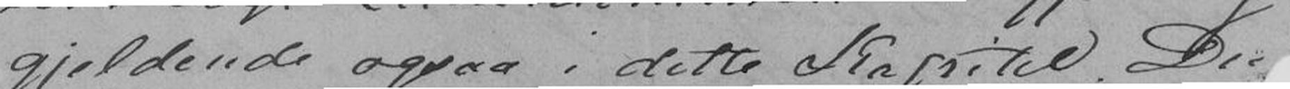gjeldende ogsaa i dette Kapitel. Du
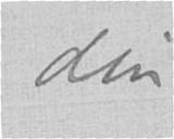din
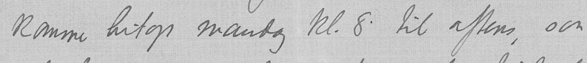komme hitop mandag kl. 8 til aftens, saa
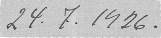24.7.1926.
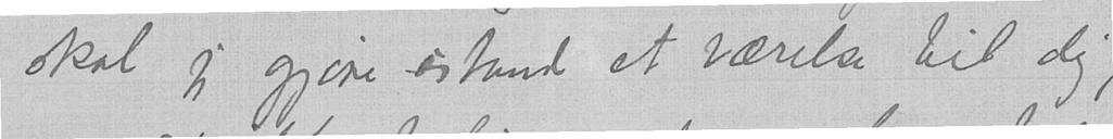skal jeg gjøre istand et værelse til dig,
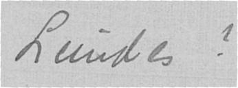Lundes?
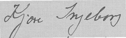Kjære Ingeborg
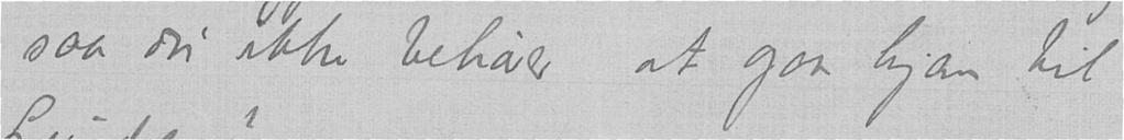saa du ikke behøver at gaa hjem til
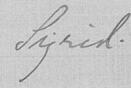Sigrid.
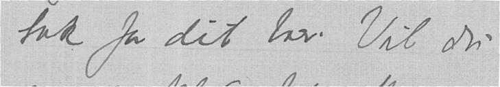tak for dit brev. Vil du
